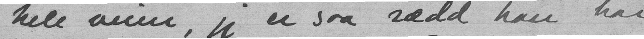hele veien, jeg er saa rædd han har
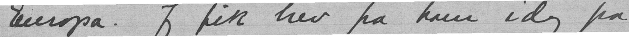Europa. Jeg fik brev fra ham i dag fra
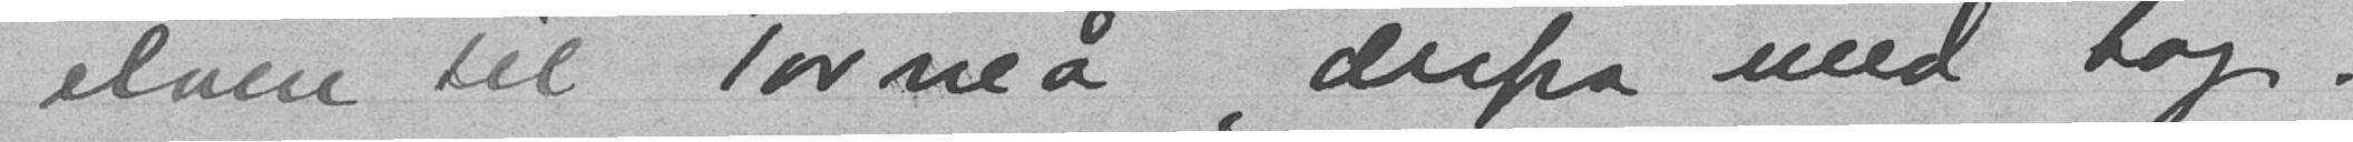elven til Torneå, derfra med tog.
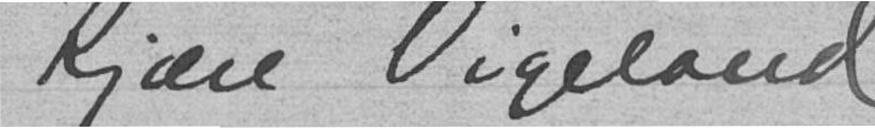Kjære Vigeland
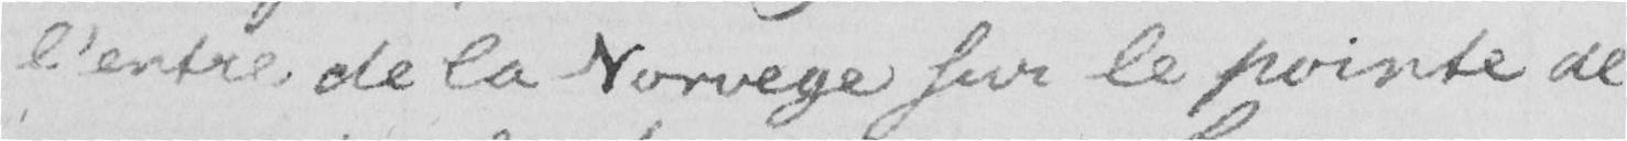l'entre de la Norvege sur le pointe de
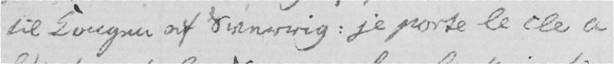til Kongen af Sverrig: je porte le cle a
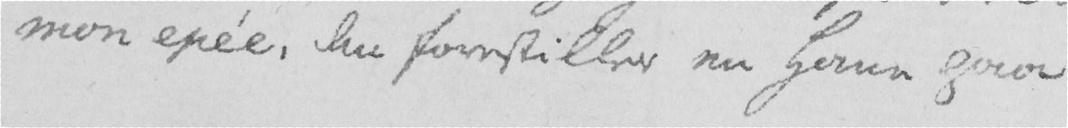mon epée, den forestiller en Hane paa
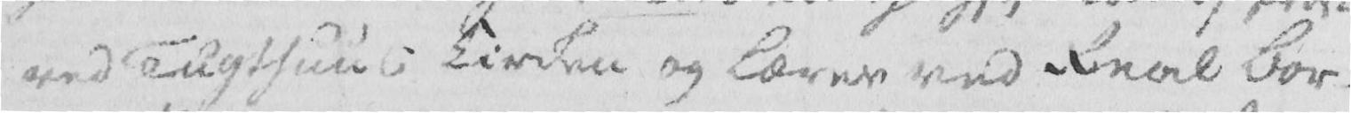ved Tugthuus Kircken og lærer ved Real Bor-
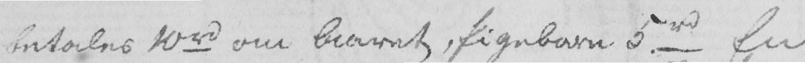betales 10 rd om Aaret, Pigebarn 5 rd En
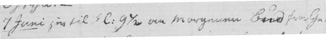7 Juni sov til Kl. 9 ½ om Morgenen Bud fra Ge-
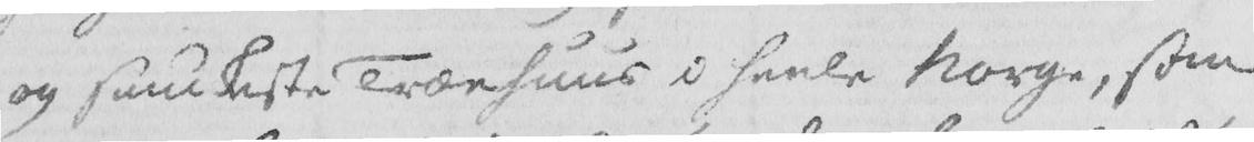og smuckeste Træehuus i heele Norge, som
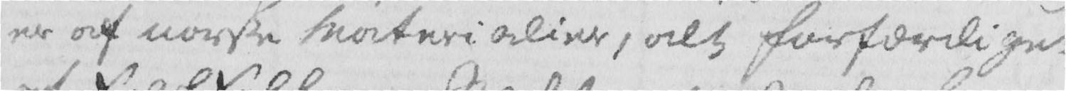er af norske Materialier, alt forfærdiget
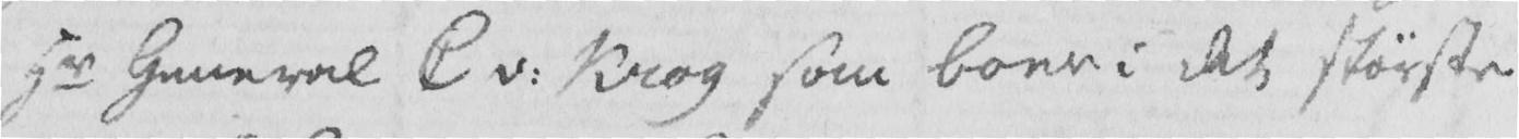Hr General G v. Krog som boer i det største
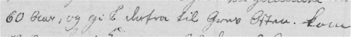60 Aar, og gik derfra til Grev Osten. Kom
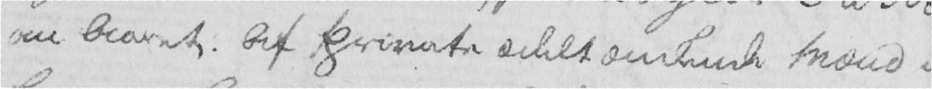om Aaret. Af Private ædeltænkende Mænd i
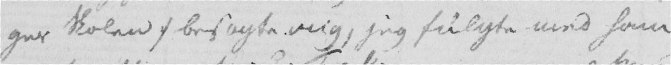ger Skolen :/ besøgte mig, jeg fulgte med ham
